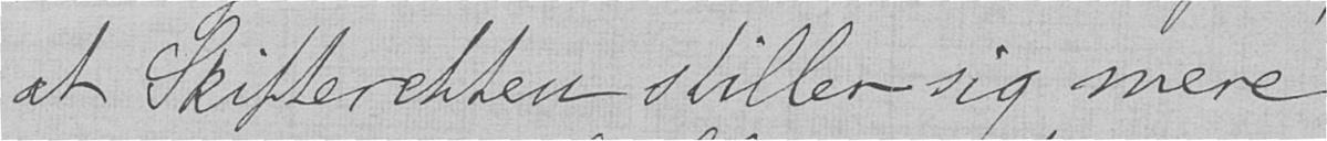at Skifteretten stiller sig mere
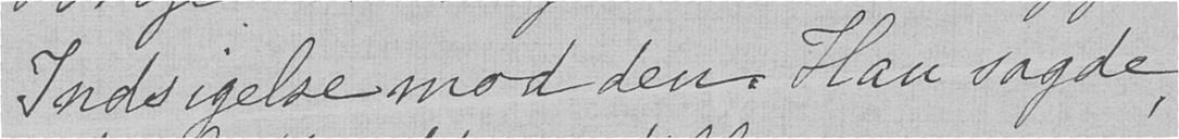Indsigelse mod den. Han sagde
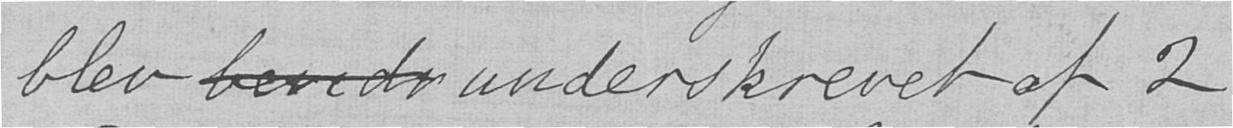blev  underskrevet af 2
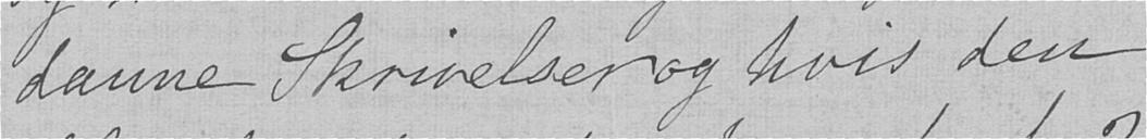danne Skrivelser og hvis den
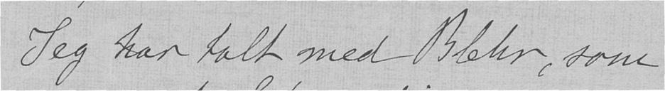Jeg har talt med Blehr, som
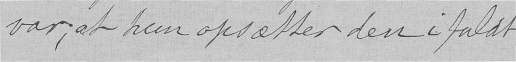var, at hun opsætter den i fuldt
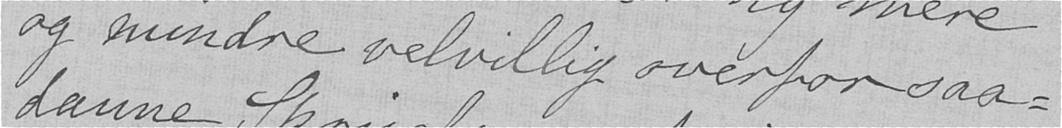og mindre velvillig overfor saa-
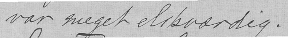var meget elskværdig.
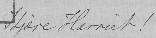Kjære Harriet!
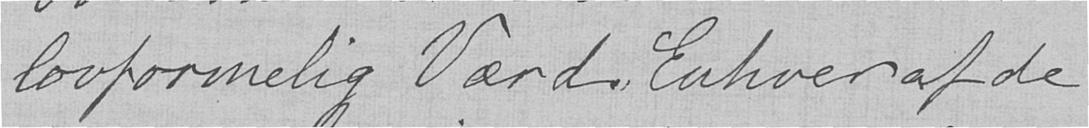lovformelig Værd. Enhver af de
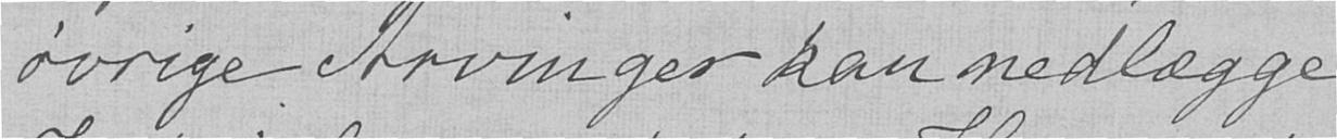øvrige Arvinger kan nedlægge
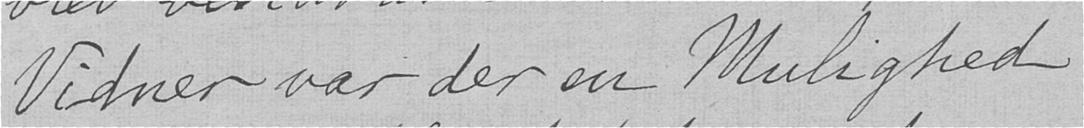Vidner var der en Mulighed
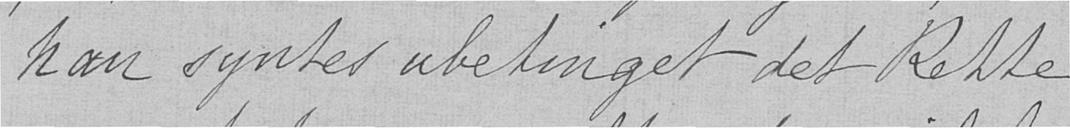han syntes ubetinget det Rette
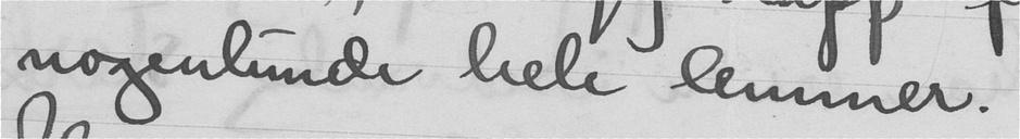nogenlunde hele lemmer.
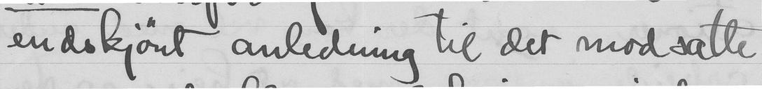endskjønt anledning til det modsatte
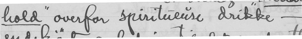hold" overfor spiritueuse drikke -
